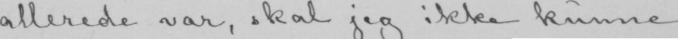allerede var, skal jeg ikke kunne
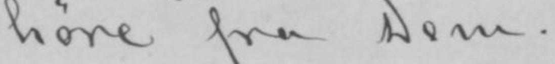høre fra Dem.
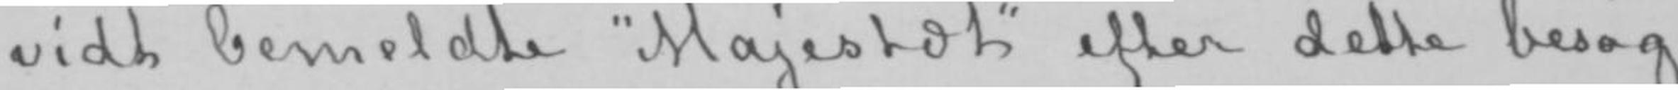vidt bemeldte «Majestæt» efter dette besøg
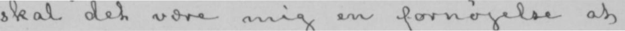skal det være mig en fornøjelse at
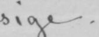sige.
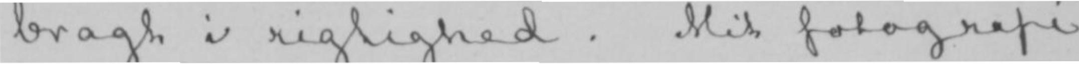bragt i rigtighed. Mit fotografi
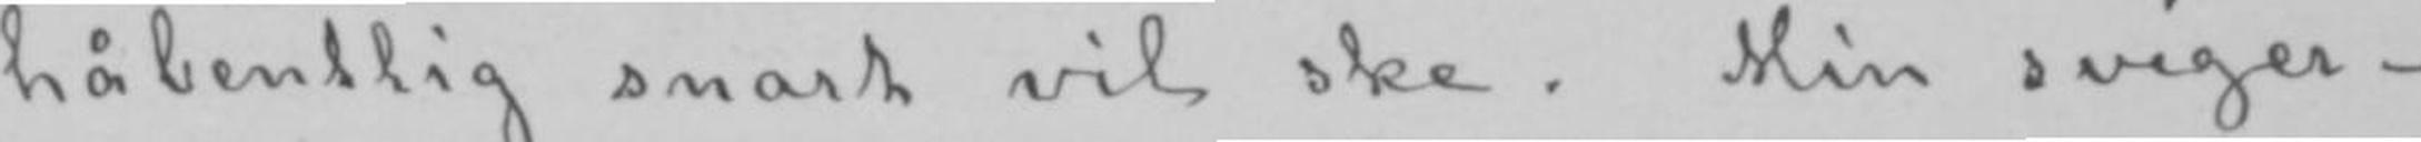håbentlig snart vil ske. Min sviger-
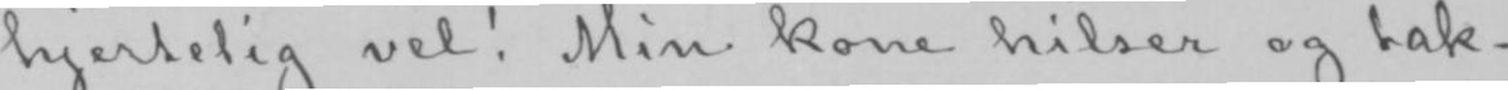hjertelig vel! Min kone hilser og tak-
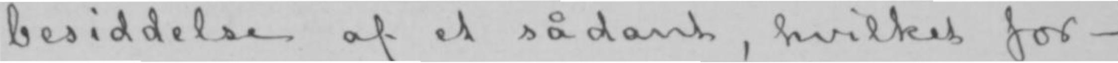besiddelse af et sådant, hvilket for-
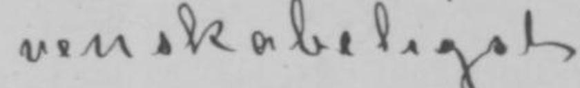venskabeligst
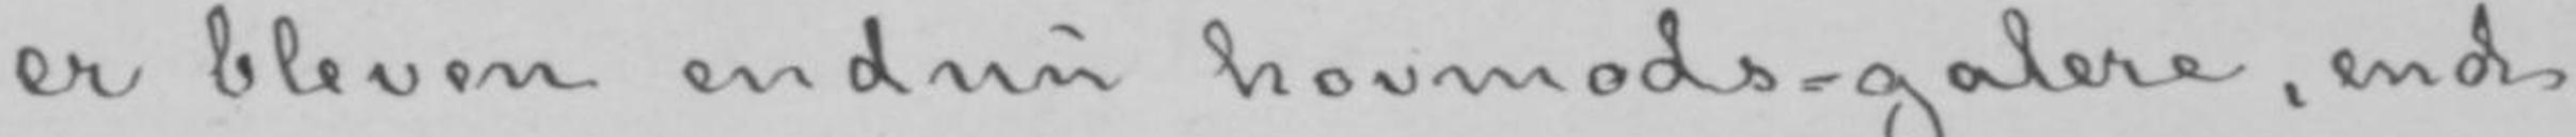er bleven endnu hovmods-galere, end
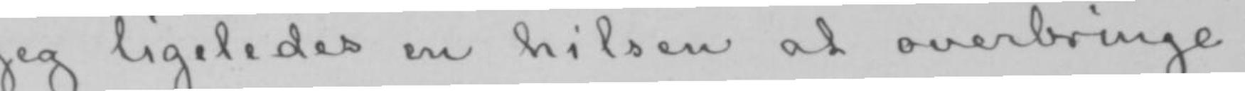jeg ligeledes en hilsen at overbringe.
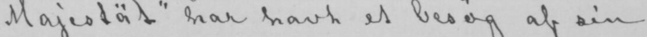Majestät» har havt et besøg af sin
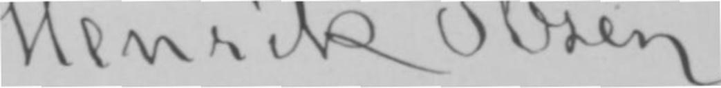Henrik Ibsen.
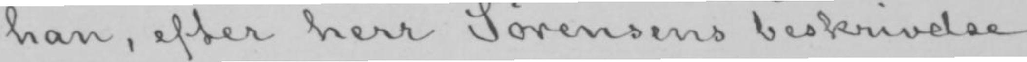han, efter herr Sørensens beskrivelse
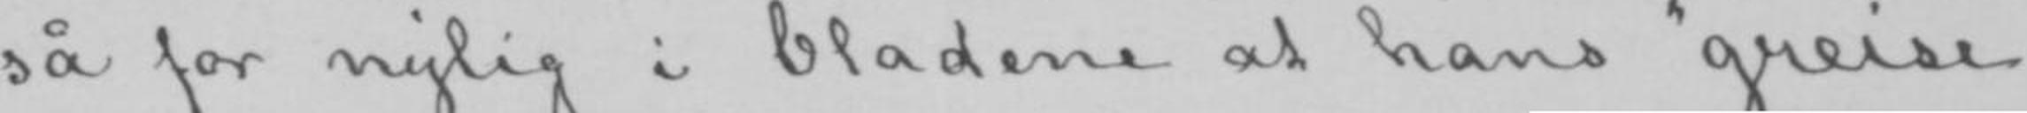så for nylig i bladene at hans «greise
